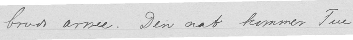bruds arme. Den nat kommer Tue
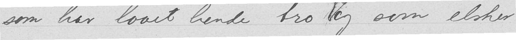som har lovet hende tro og som elsker
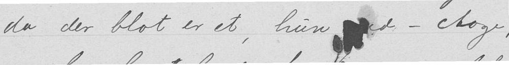da der blot er et, hun ved – Aage,
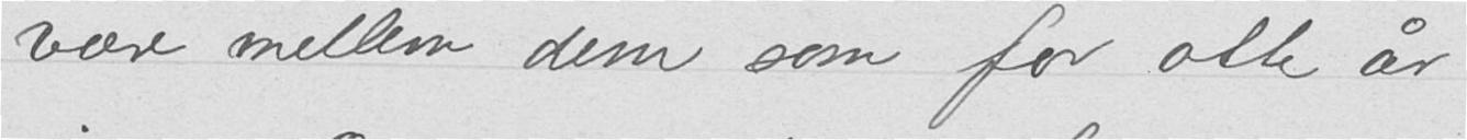være mellem dem som for otte år
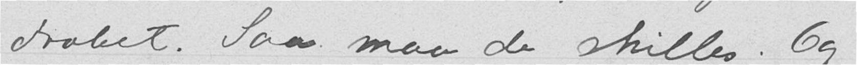drabet. Saa maa de skilles. Og
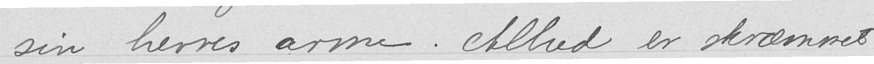sin herres arme. Alhed er skræmmet
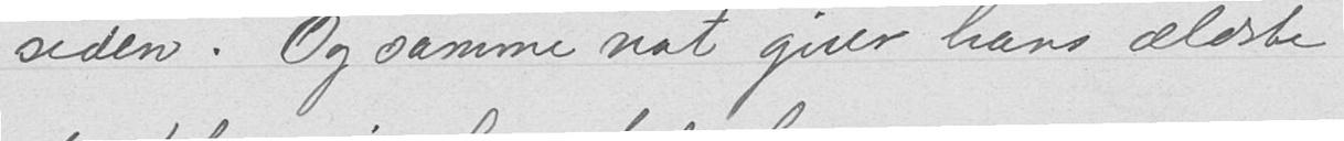siden. Og samme nat giver hans ældste
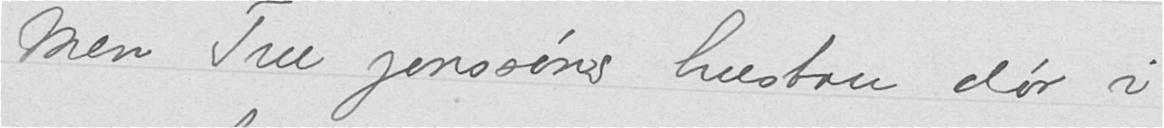Men Tue Jonssøns hustru dør i
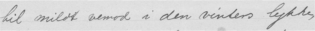til mildt vemod i den vinters lykke,
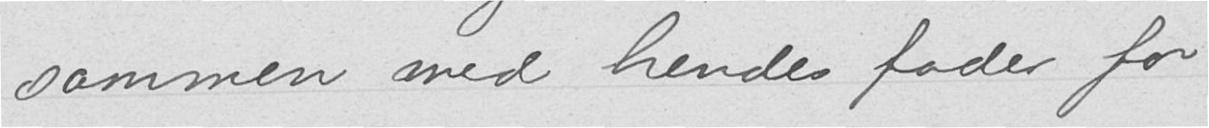sammen med hendes fader for
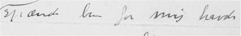spænde ben for mig havde
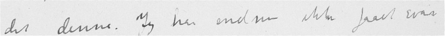det denne. Jeg har endnu ikke faaet svar
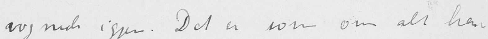vognede igjen. Det er som om alt har
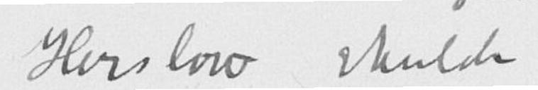Herslow skulde
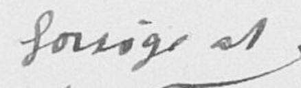forsøge at
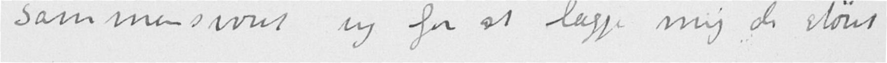sammensvoret sig for at lægge mig de størst
